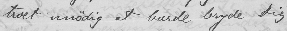troet unødig at burde bryde Dig
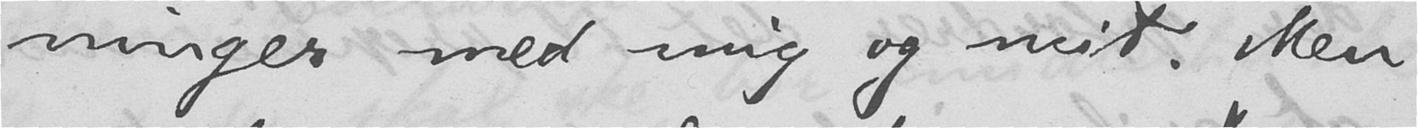ninger med mig og mit. Men
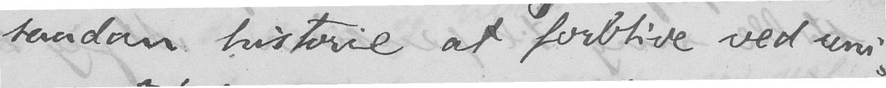saadan historie at forblive ved uni-
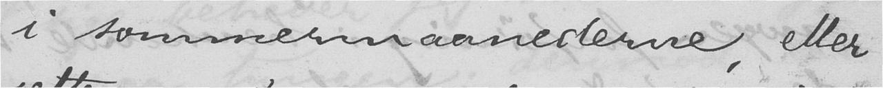i sommermaanederne, eller
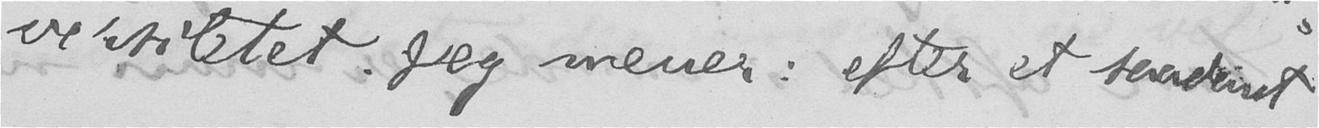versitetet. Jeg mener: efter et saadant
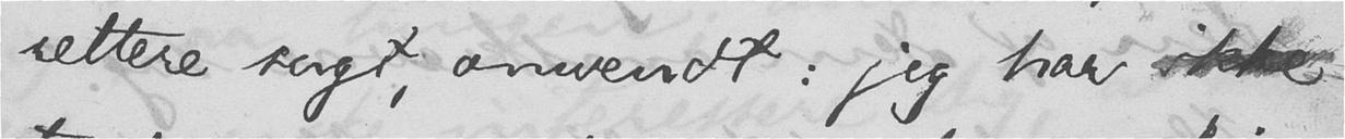rettere sagt, omvendt: jeg har ikke
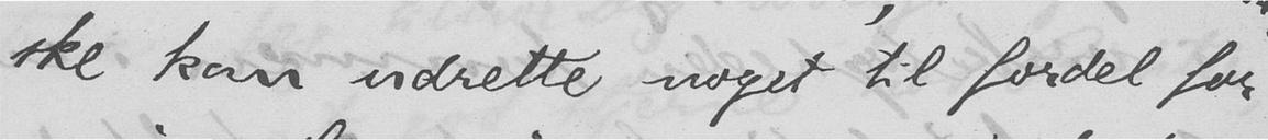ske kan udrette noget til fordel for
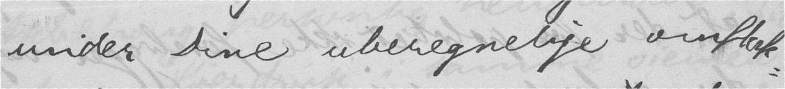under Dine uberegnelige omflak-
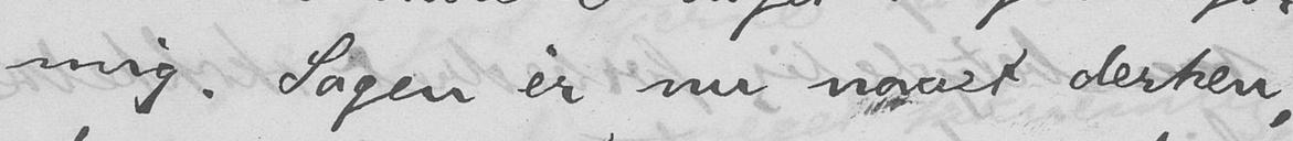mig. Sagen er nu naaet derhen,
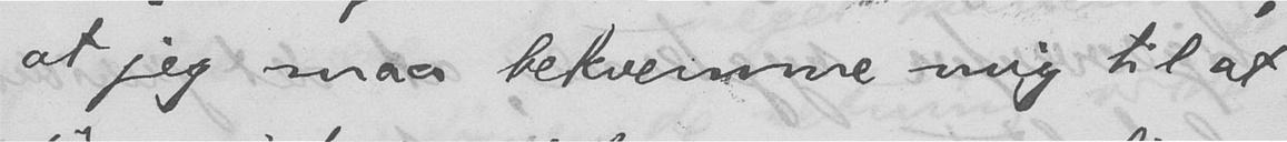at jeg maa bekvemme mig til at
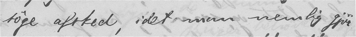søge afsked, idet man nemlig gjør
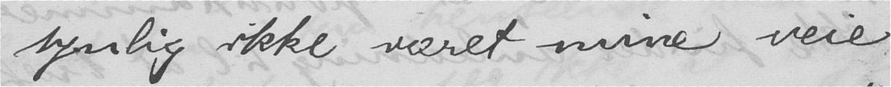synlig ikke været mine veie
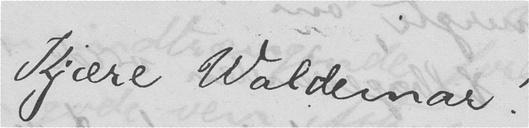Kjære Waldemar!
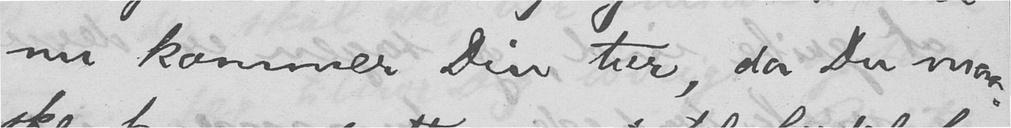nu kommer Din tur, da Du maa-
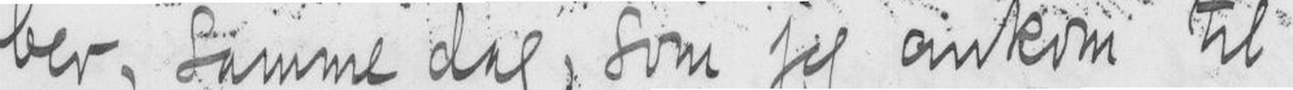ber, samme dag, som jeg ankom til
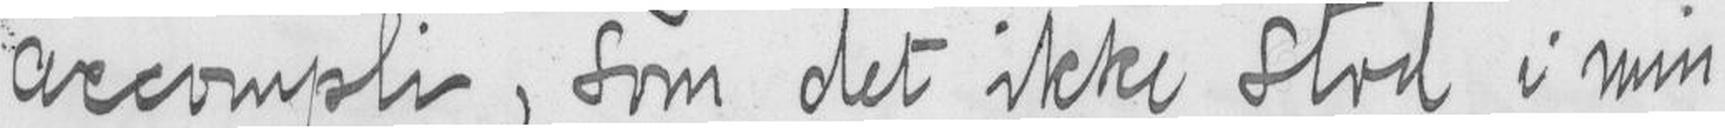accompli, som det ikke stod i min
